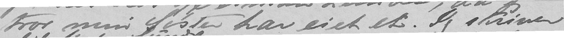tror min Søster har eiet et. Jeg skriver
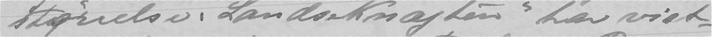størrelse. Landseknægten " har vist-
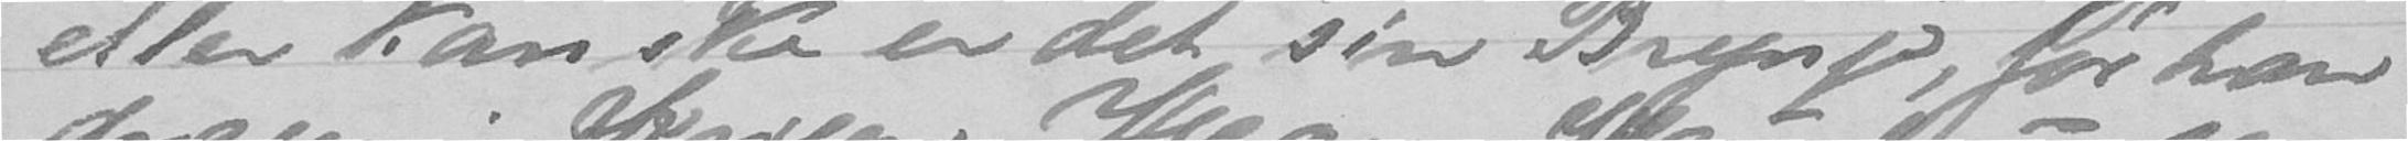eller kanske er det sin Brynje, før han
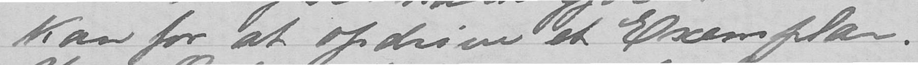kan for at opdrive et Exemplar.
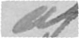af
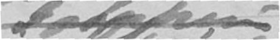Lappen
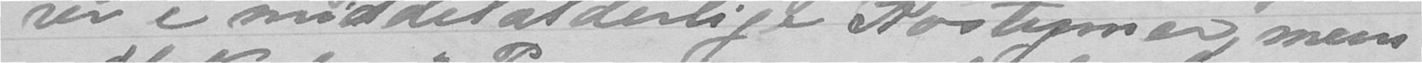rer i middelalderlige Kostymer, mens
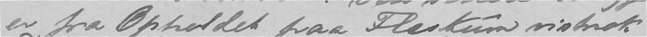er fra Opholdet paa Fleskum vistnok
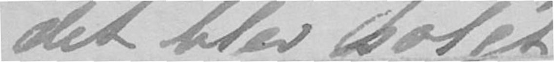det blev solgt
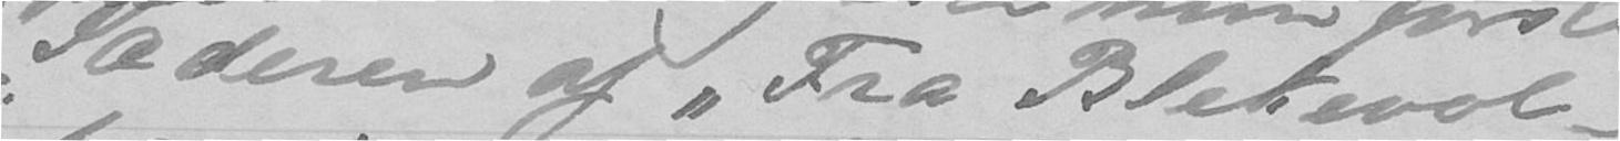Sæderen af "Fra Blekevol-
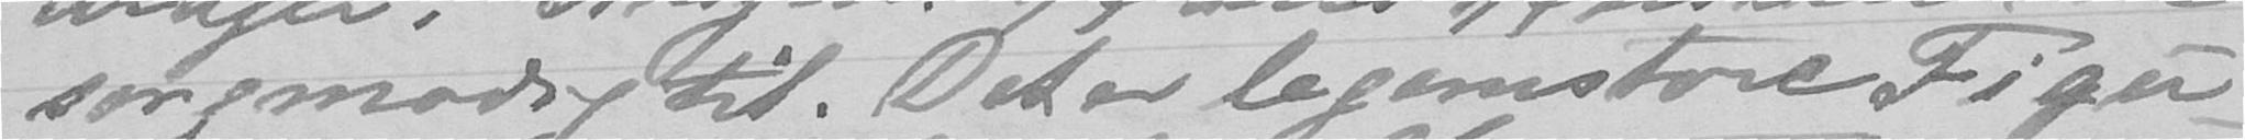sorgmodig til. Det er legemstore Figu-
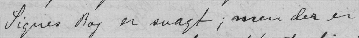Signes Bog er svagt; men der er
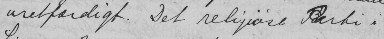uretfærdigt. Det religeøse Parti i
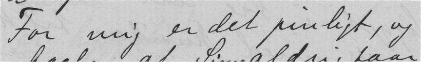For mig er det pinligt, og
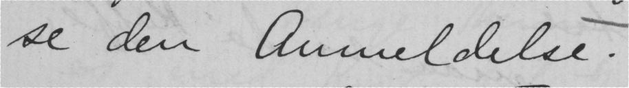se den anmeldelse.
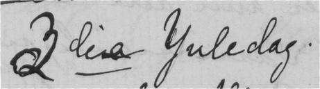3 die juledag.
